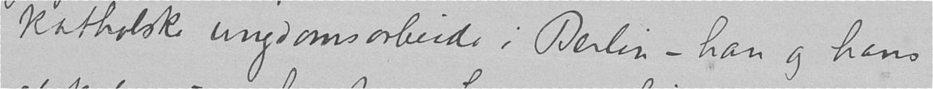katholske ungdomsarbeide i Berlin – han og hans
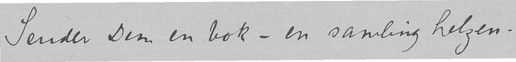Sender Dem en bok - en samling helgen-
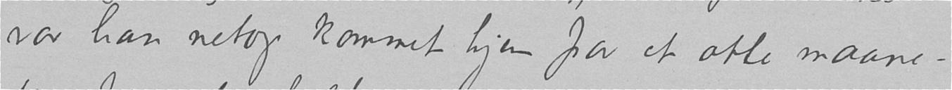var han netop kommet hjem fra et otte maane-
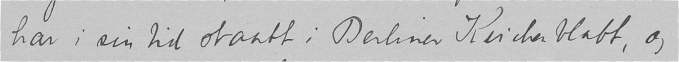har i sin tid staatt i Berliner Kirchenblatt, og
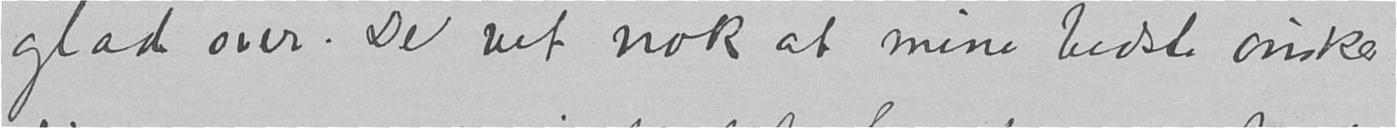glad over. De vet nok at mine bedste ønsker
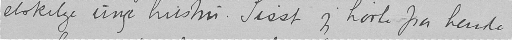elskelige unge hustru. Sisst jeg hørte fra hende
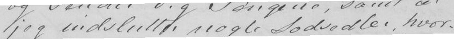jeg indslutter nogle Lodsedler. hvor
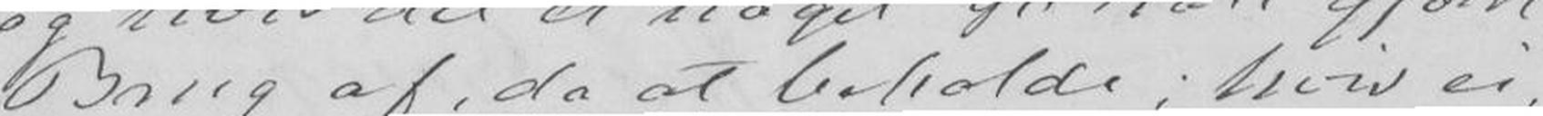Brug af, da at beholde; hvis ei,
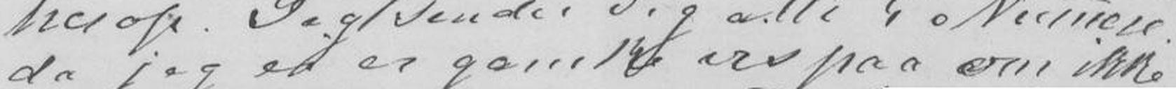da jeg ei er ganske vis paa om ikke
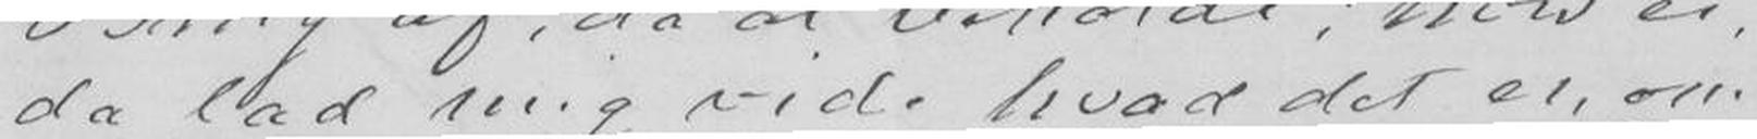da lad mig vide hvad det er, om
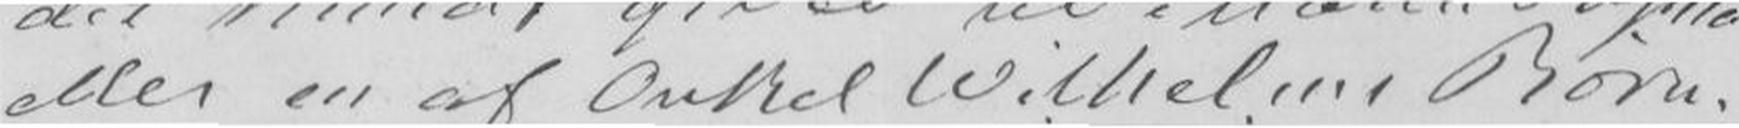eller en af Onkel Wilhelms Børn,
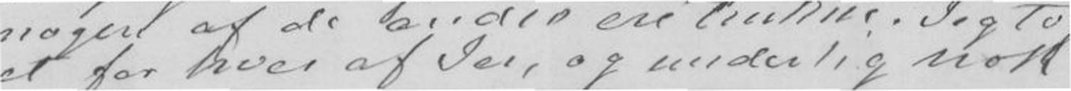et for hver af jer, og underlig nok
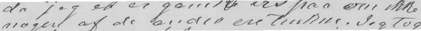nogen af de andre ere trukne. Jeg tog
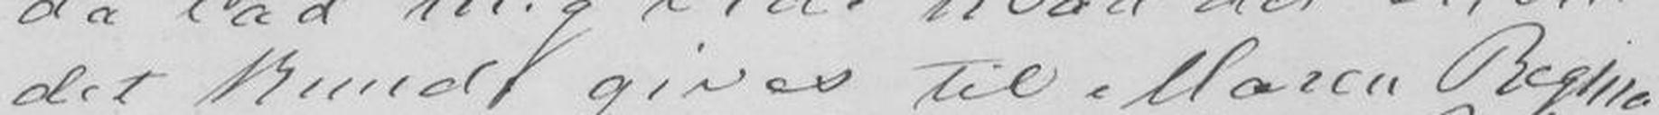det kunde gives til Maren Regine
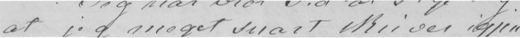at jeg meget snart skriver igjen
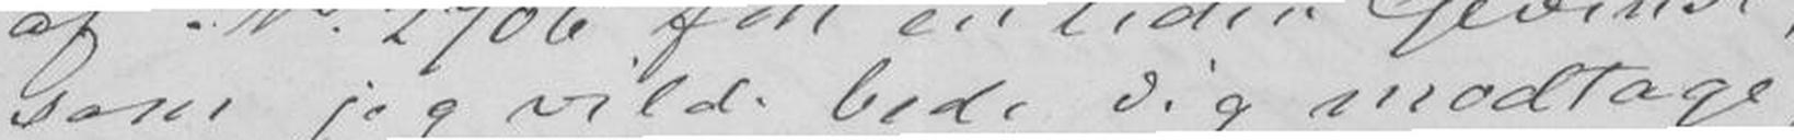som jeg vilde bede Dig modtage,
